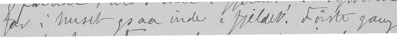far i huset ogsaa inde i fjeldet! Første gang
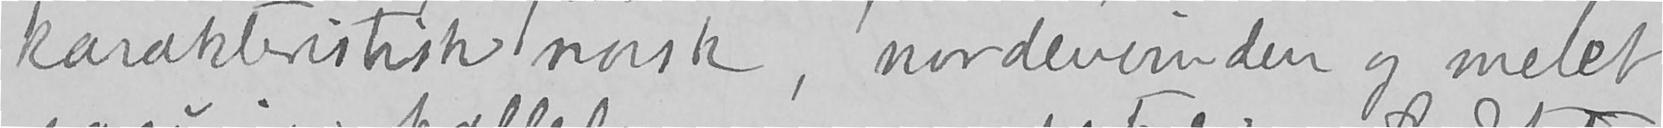karakteristisk norsk, nordenvinden og melet
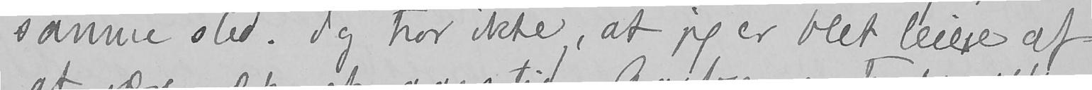samme sted. Jeg tror ikke, at jeg er blet leiere af
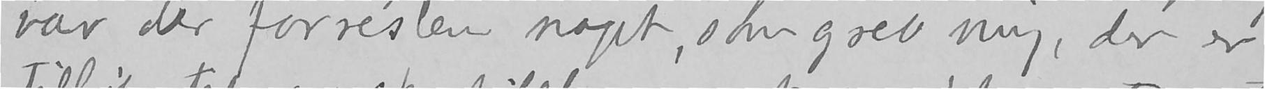var der forresten noget, som greb mig, der er
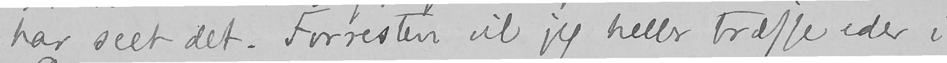har seet det. Forresten vil jeg heller træffe eder i
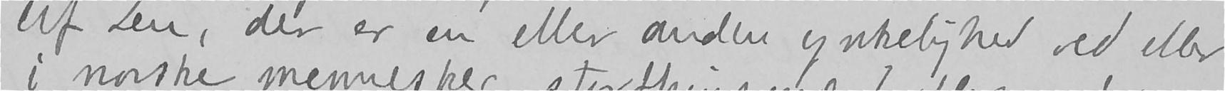Uf Du, der er en eller anden ynkelighed ved eller
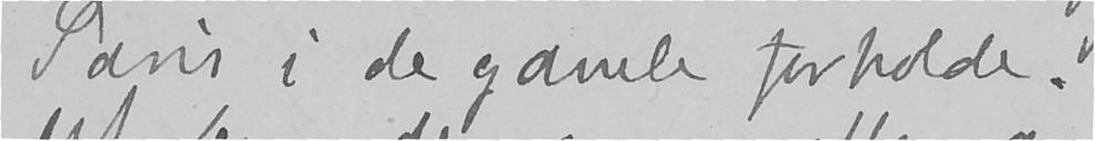Paris i de gamle forholde.
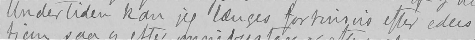Undertiden kan jeg længes fortrinsvis efter eders
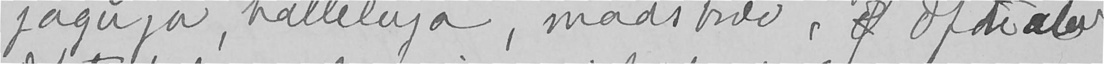jagu ja, halleluja, maalstræv, Ø Ofdealen
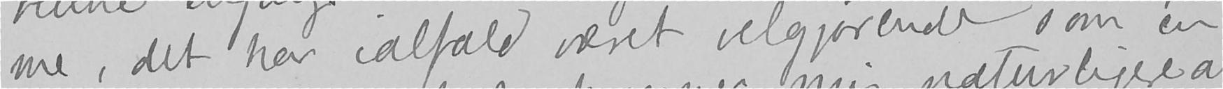me, det har ialfald været velgjørende, som en
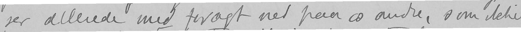ser allerede med foragt ned paa os andre, som ikke
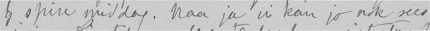og spise middag. Naa ja vi kan jo nok sees
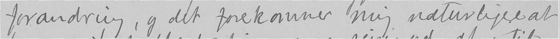forandring, og det forekommer mig naturligere at
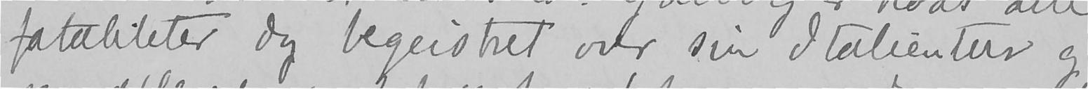fataliteter dog begeistret over sin Italientur og
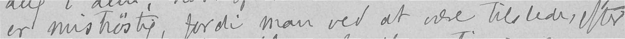er mistrøstig, fordi man ved at være tilstede, efter
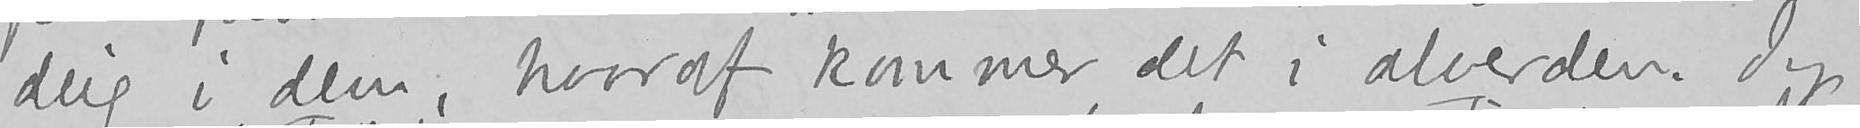deig i dem, hvoraf kommer det i alverden. Jeg
